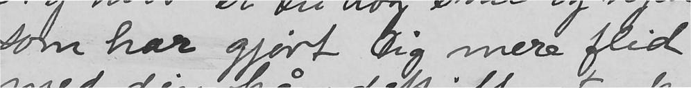som har gjort Dig mere flid
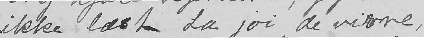ikke læst La joie de vivre,
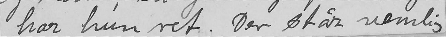har hun ret. Der står nemlig
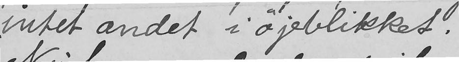intet andet i øjeblikket.
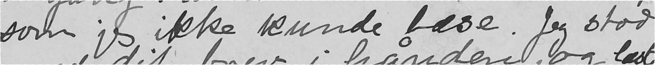som jeg ikke kunde læse. Jeg stod
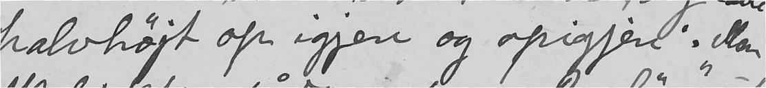halvhøjt op igjen og opigjen: "Man
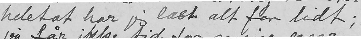heletat har jeg læst alt for lidt;
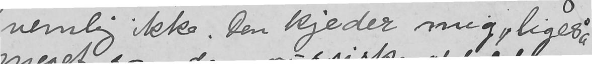nemlig ikke. Den kjeder mig, ligeså
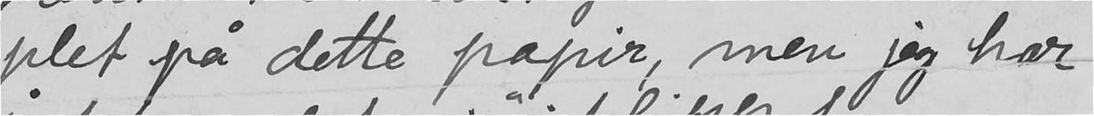plet på dette papir, men jeg har
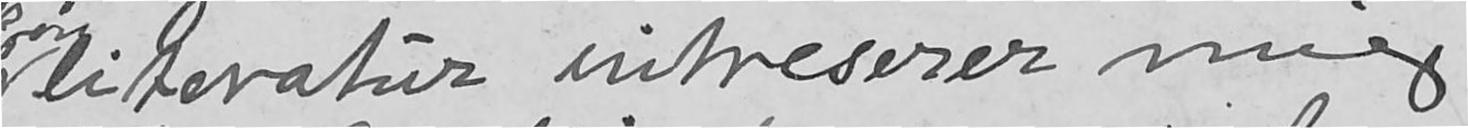literatur intreserer mig
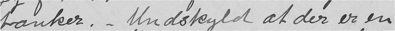tanker. - Undskyld at der er en
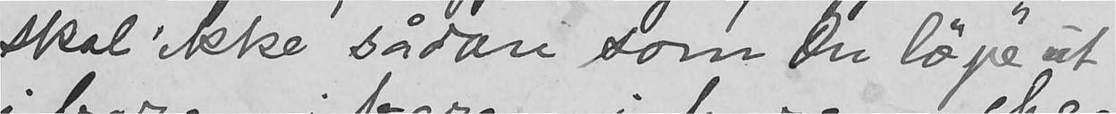skal ikke sådan som Du løpe ut
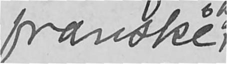franske
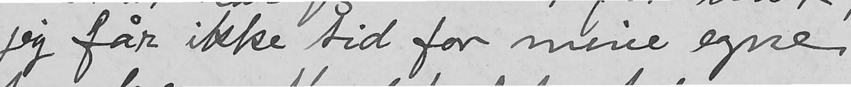jeg får ikke tid for mine egne
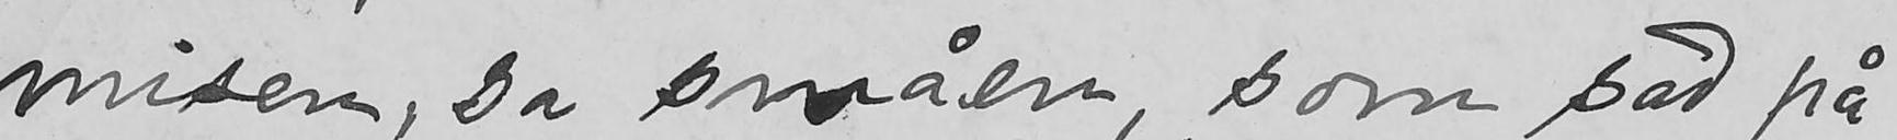misen, sa småen, som sad på
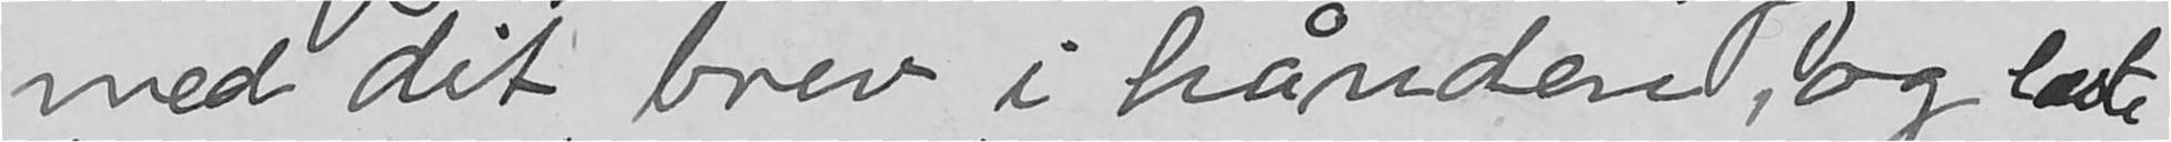med dit brev i hånden, og læste
